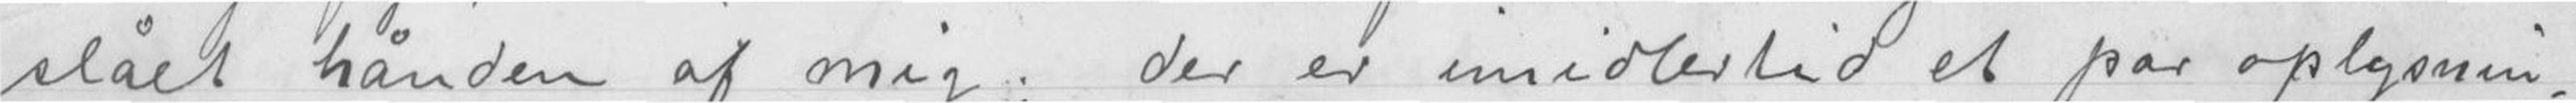slået hånden af mig; Der er imidlertid et par oplysning-
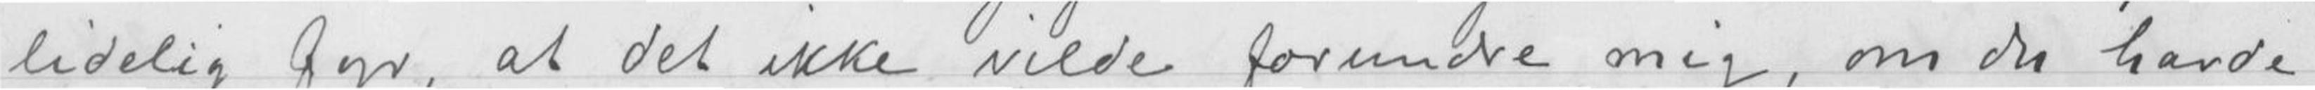lidelig fyr, at det ikke vilde forundre mig, om du havde
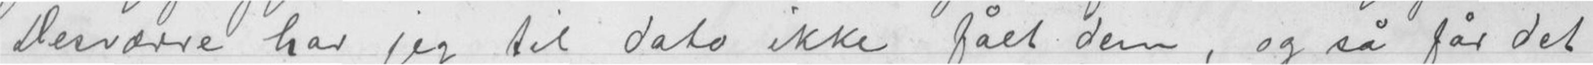Desværre har jeg til dato ikke fået dem, og så får det
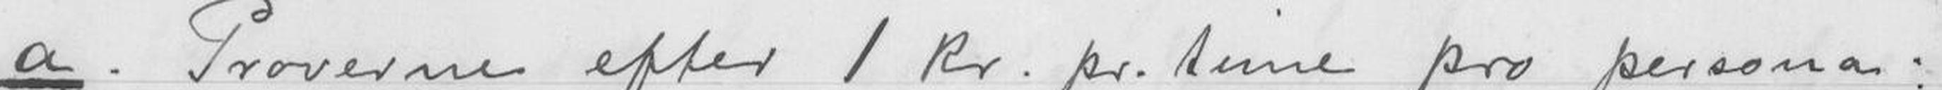a. Proverne efter 1 kr. pr. time pro persona:
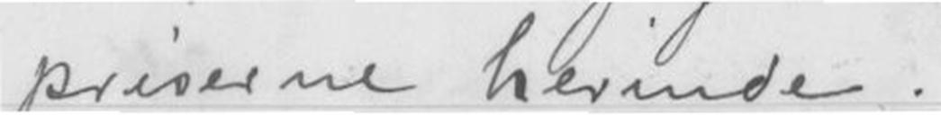priserne herinde.
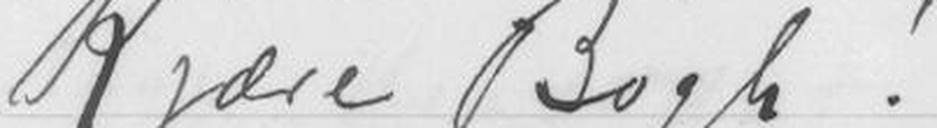Kjære Bøgh!
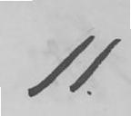11.
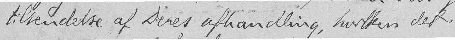tilsendelse af Deres afhandling, hvilken det
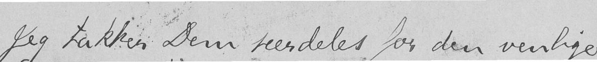Jeg takker Dem særdeles for den venlige
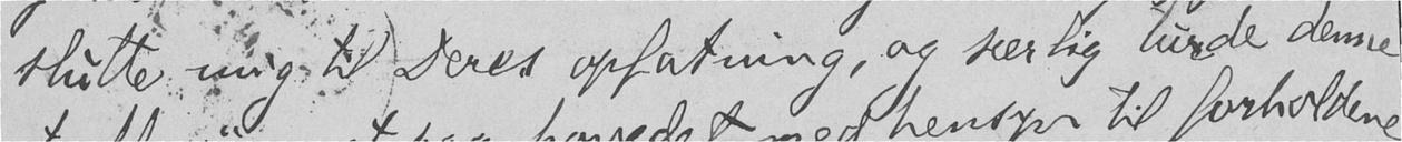slutte mig til Deres opfatning, og særlig turde denne
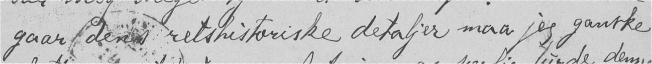gaar dens retshistoriske detaljer maa jeg ganske
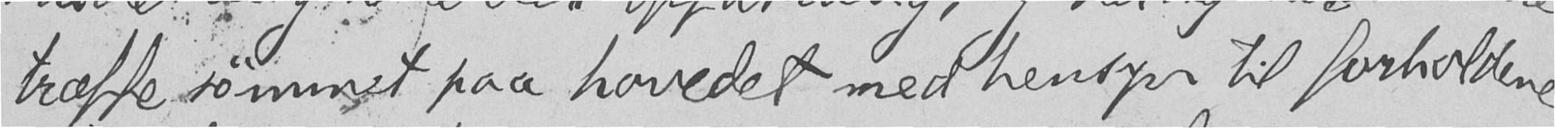træffe sømmet paa hovedet med hensyn til forholdene
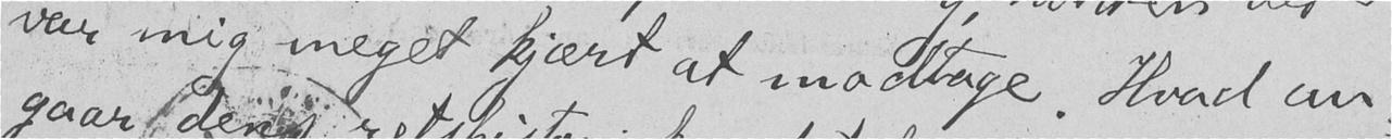var mig meget kjært at modtage. Hvad an-
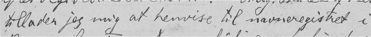tillader jeg mig at henvise til navneregistret i
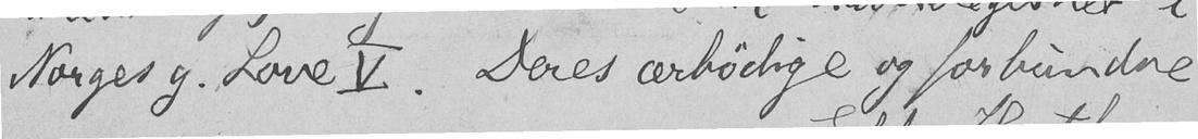Norges g. Love V. Deres ærbødige og forbundne
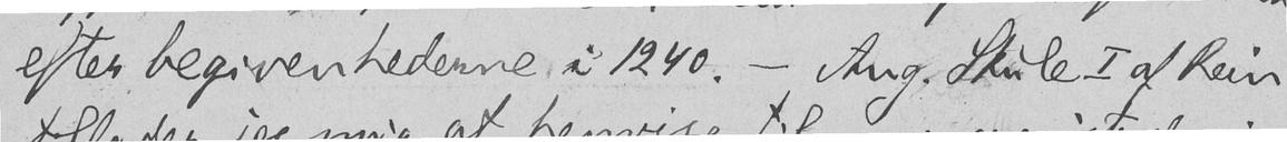efter begivenhederne i 1240. – Ang. Skule I af Rein
